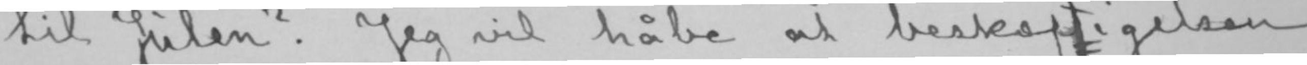til Julen? Jeg vil håbe at beskæfftigelsen
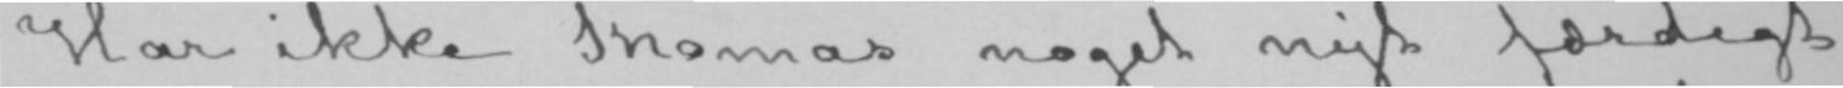Har ikke Thomas noget nyt færdigt
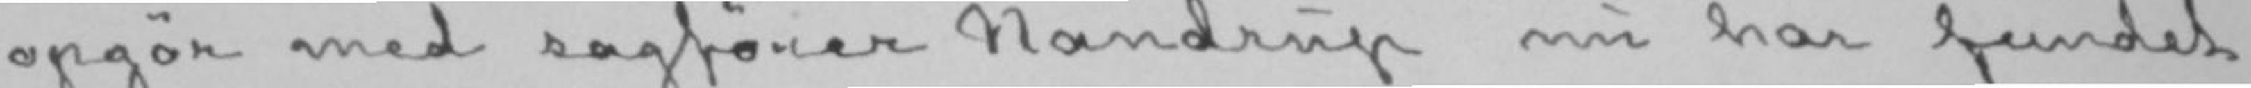opgør med sagfører Nandrup nu har fundet
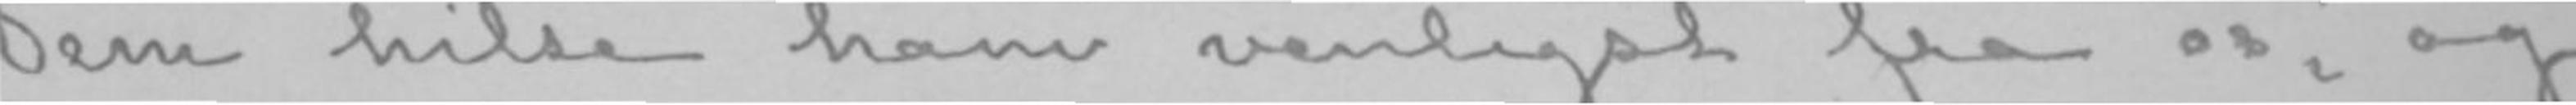Dem hilse ham venligst fra os, og
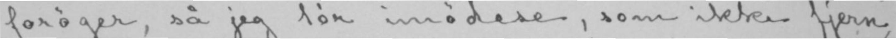forøger, så jeg tør imødese, som ikke fjern,
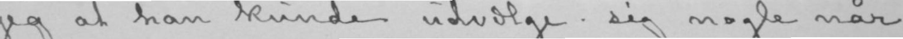jeg at han kunde udvælge sig nogle når
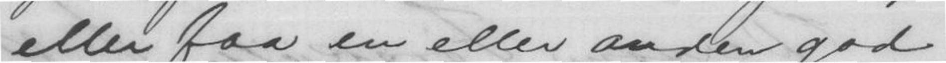eller faa en eller anden god
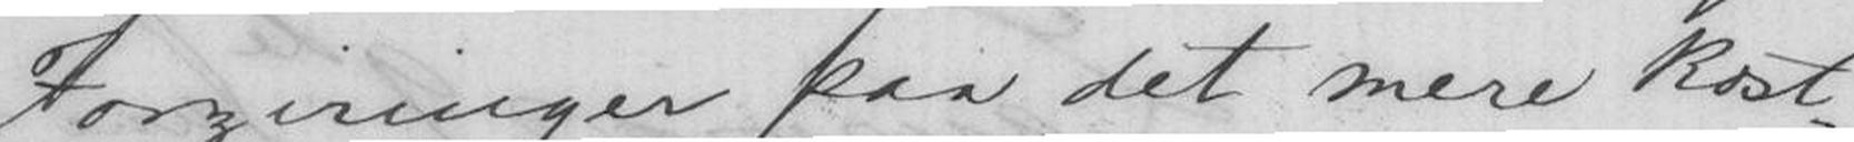Forziringer paa det mere Kost-
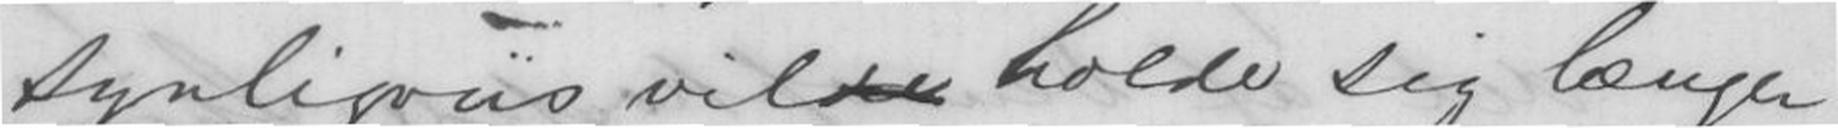synligvis vil holde sig længer
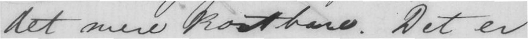det mere kostbare. Det er
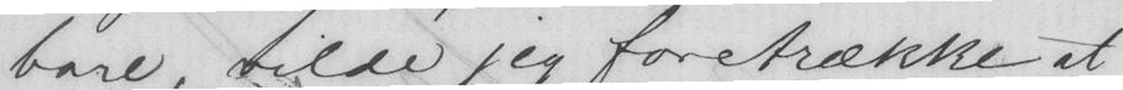bare, vilde jeg foretrække at
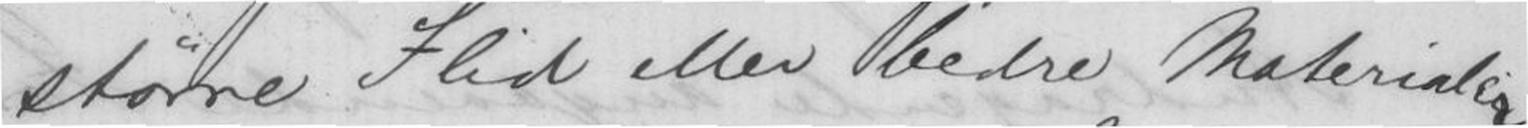større Flid eller bedre Materialer
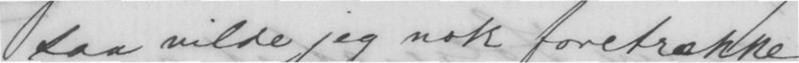saa vilde jeg nok foretrække
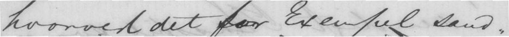hvorved det for Exenpel sand-
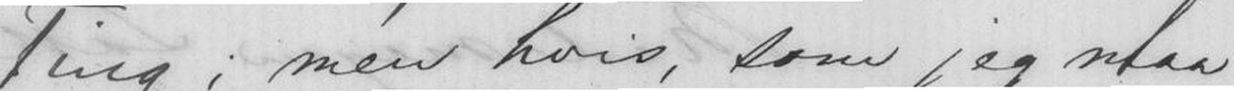Ting; men hvis, som jeg maa
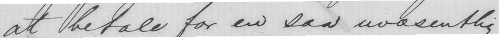at betale for en saa uvæsentlig
